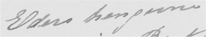Eders hengivne
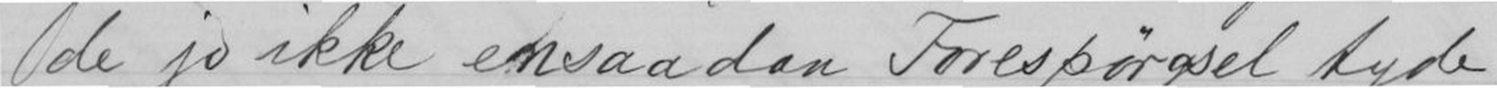de jo ikke en saaden Forespørgsel tyde
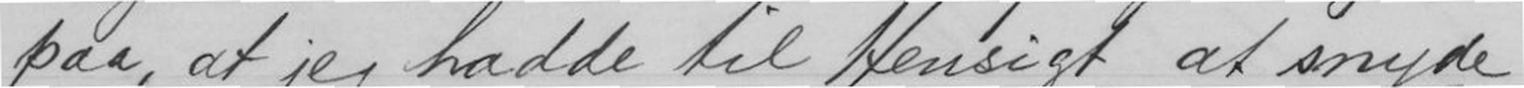paa, at jeg hadde til Hensigt at snyde
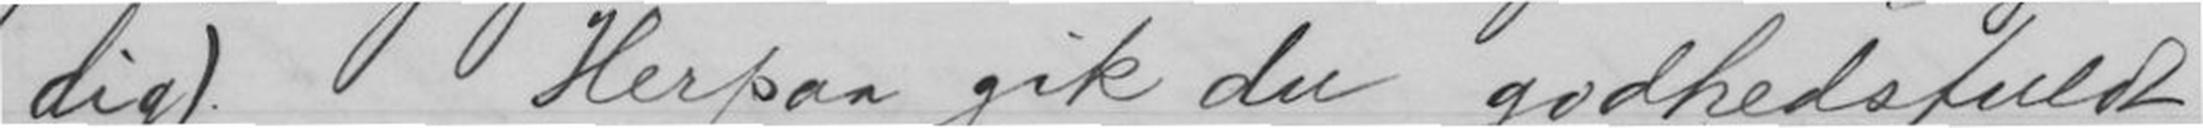dig). Herpaa gik du godhedsfuldt
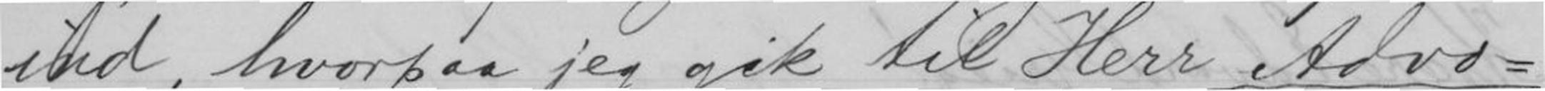ind, hvorpaa jeg gik til Herr Advo-
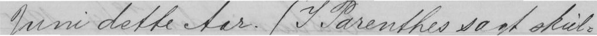Juni dette Aar. (I Parenthes sagt skul-
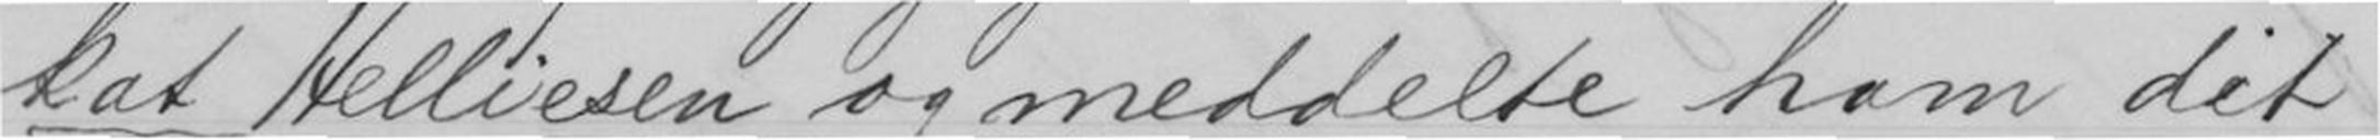kat Helliesen og meddelte ham dit
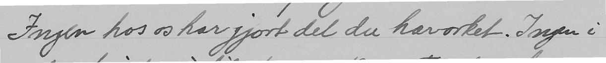Ingen hos os har gjort det du hav orket. Ingen i
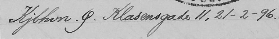Kjbhvn. Ø. Klasensgade 11. 21-2-96.
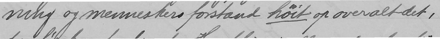ning og menneskers forstand høit op over alt det,
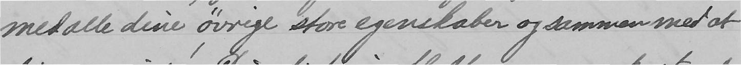med alle dine øvrige stor egenskaber og sammen med at
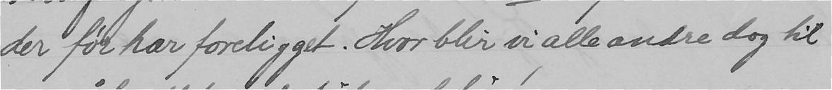der før har foreligget. Hvor blir vi alle andre dog til
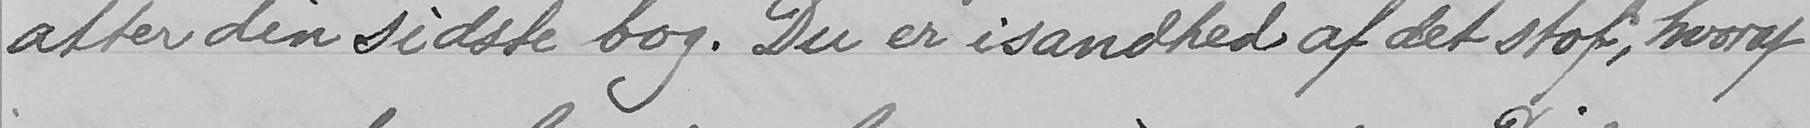atter din sidste bog. Du er isandhed af det stof, hvoraf
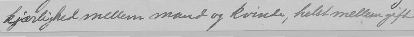kjærlighed mellem mand og kvinde, helst mellem gift
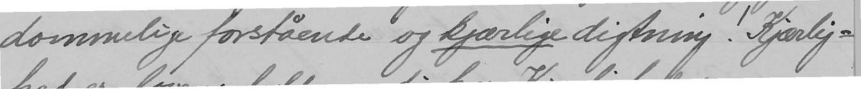dommelige forstående, og kjærlige digtning. Kjærlig-
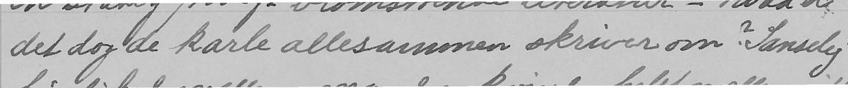det dog de karle allesammen skriver om? Sanselig
